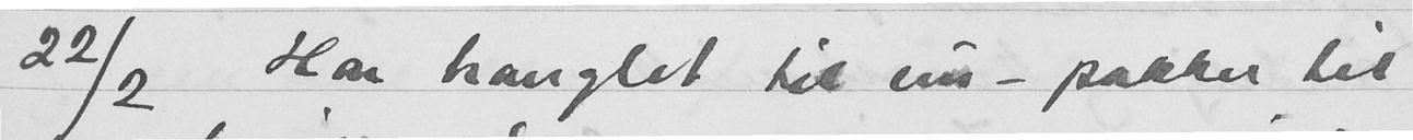22/2 Har kranglet til nu-pakker til
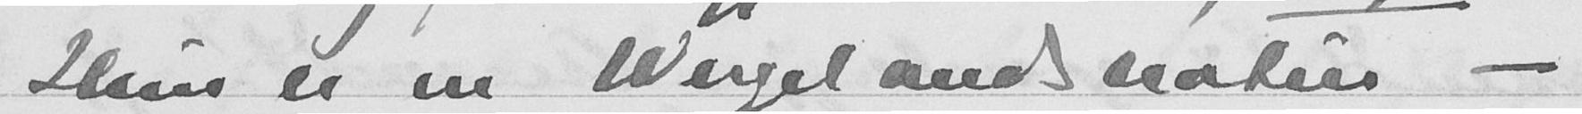Hun er en Wergelandsnatin -
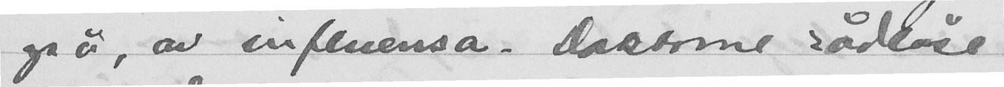også, av influensa. Doktorne rådlase
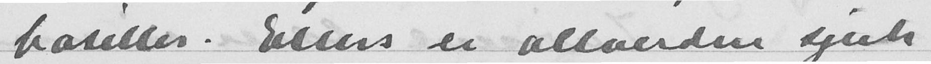basillen. Ellers er allverden sjuk
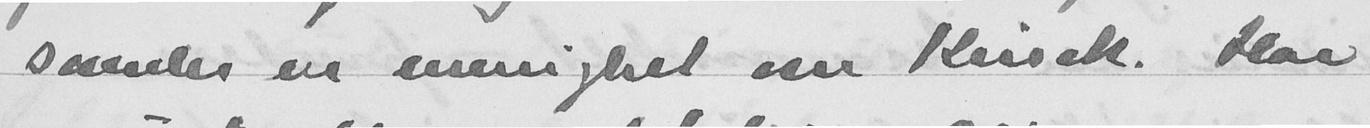samler en menighet om Kinck. Har
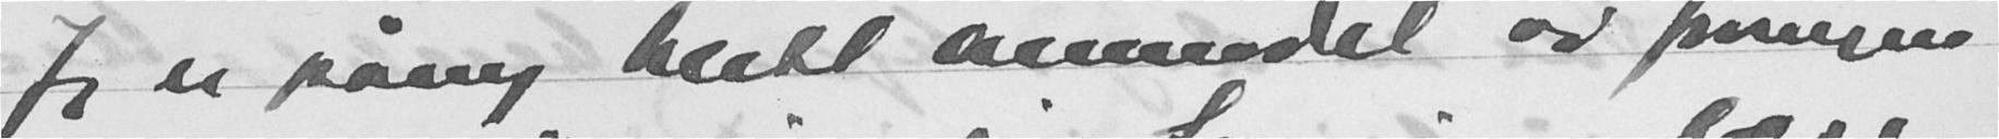Jeg er påny blitt anmodet av foreningen
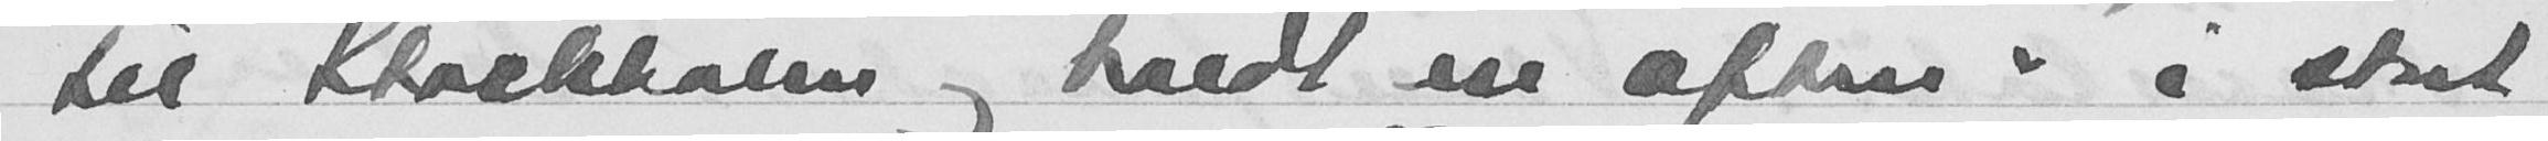til Stockholm og holdt "en aften" i stort
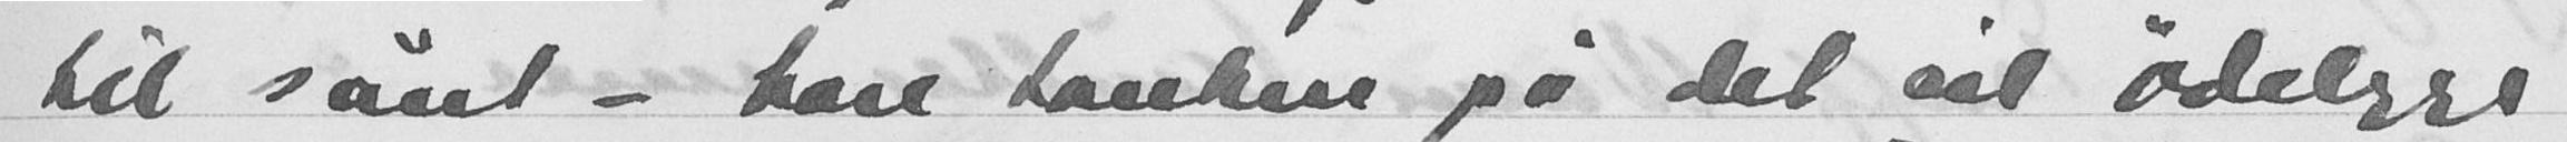til sånt - bare tanken på det vil ødelegge
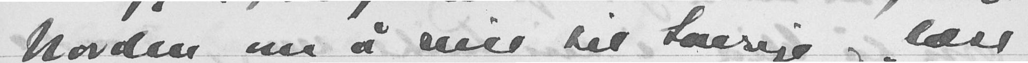Norden om å reise til Sverige "læse
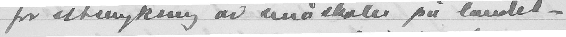for utsmykning av småskoler på landet -
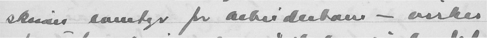skriver eventyr for arbeiderbarn - virker
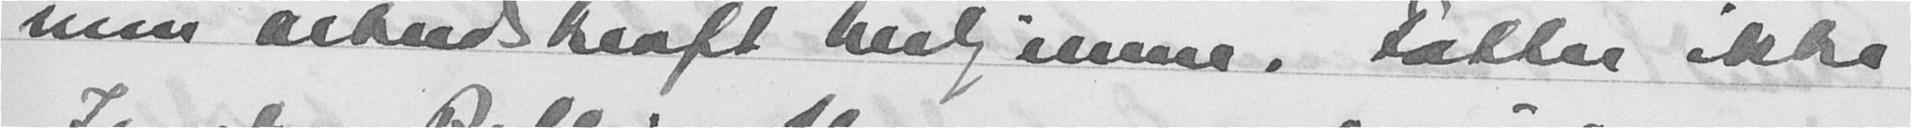min arbeidskraft her hjemme. Fatter ikke
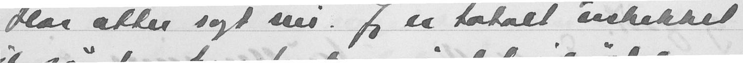Har atter sagt nei. Jeg er totalt uskikket
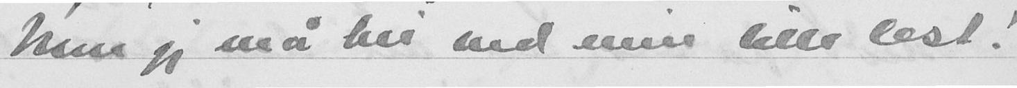Men jeg må tre av min lille lest!
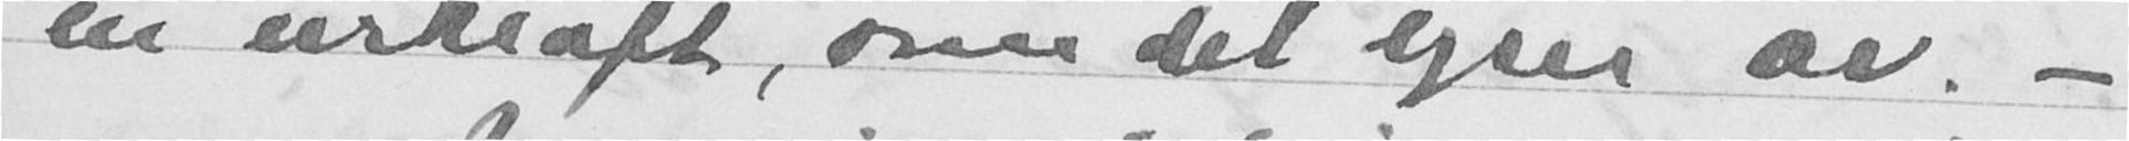en urkraft, som det lyser av. -
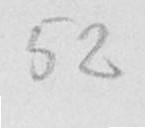52
